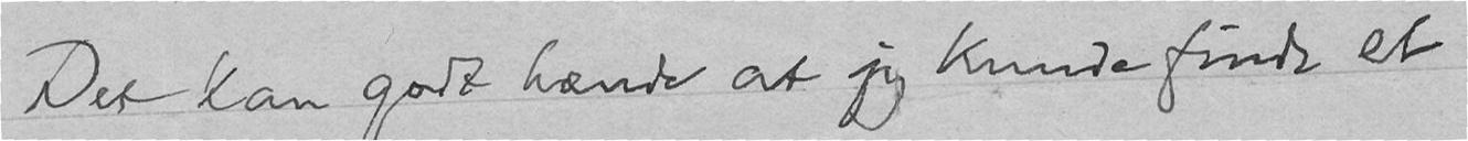Det kan godt hænde at jeg kunde finde et
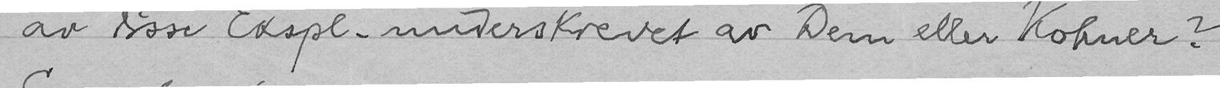av disse Ekspl. underskrevet av Dem eller Kohner?
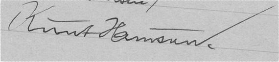Knut Hamsun.
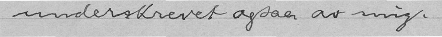underskrevet ogsaa av mig.
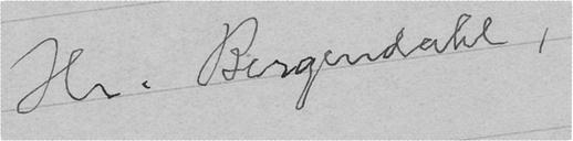Hr. Bergendahl,
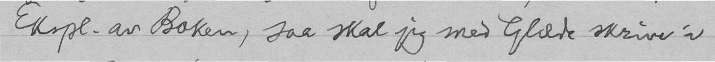Ekspl. av Boken, saa skal jeg med Glæde skrive i
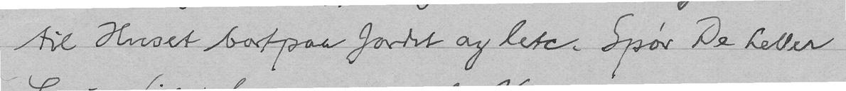til Huset bortpaa Jordet og lete. Spør De heller
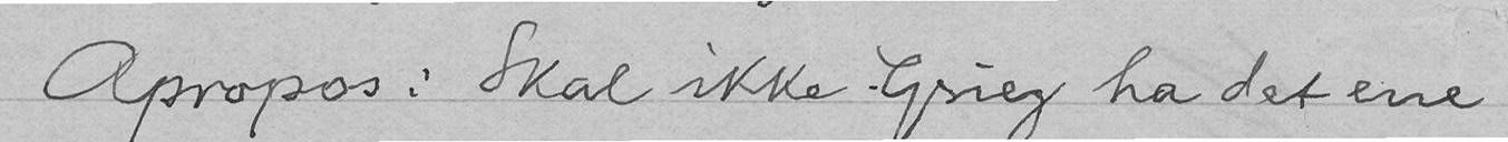Apropos: Skal ikke Grieg ha det ene
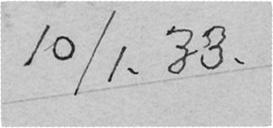10/1. 33.
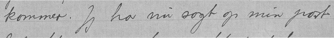kommer. Jeg har nu sagt op min post
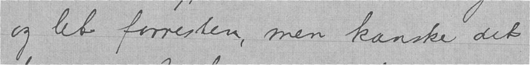og let forresten, men kanske det
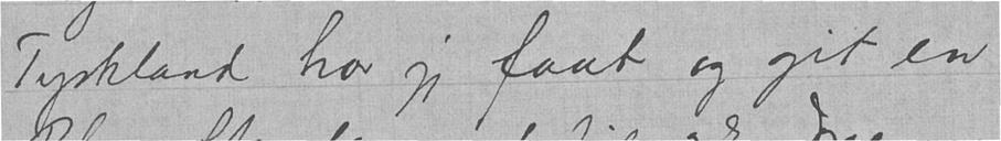Tyskland har jeg faat og git en
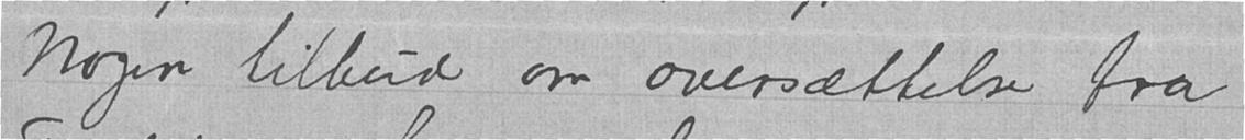Nogen tilbud om oversættelse fra
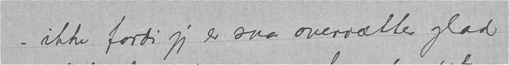- ikke fordi jeg er saa overvættes glad
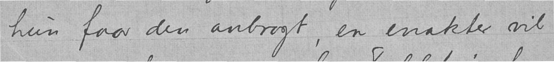hun faar den anbragt, en enakter vil
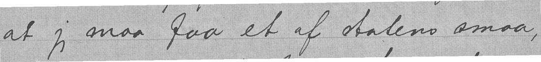at jeg maa faa et af statens smaa,
